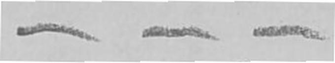- - -
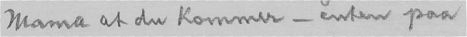Mama at du kommer - enten paa
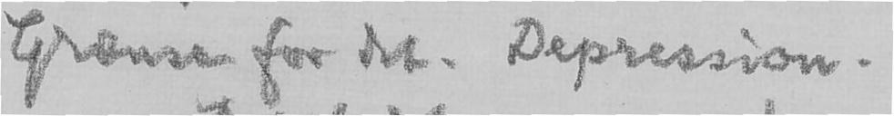Grænser for det. Depression.
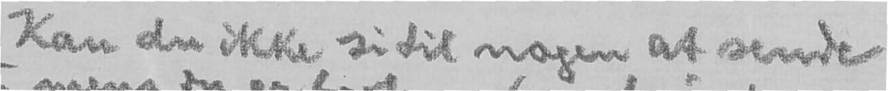Kan du ikke si til nogen at sende
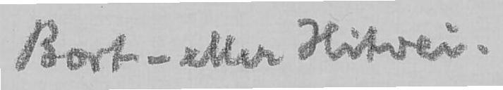Bort- eller Hitvei.
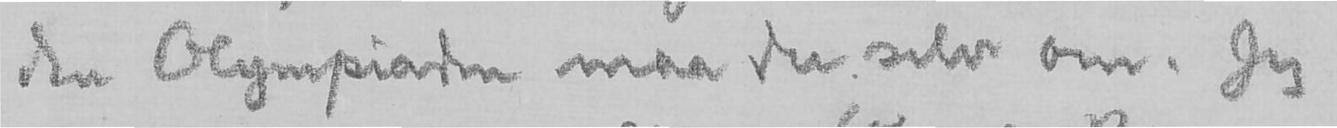den Olympiaden maa du selv om. Jeg
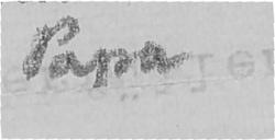Papa
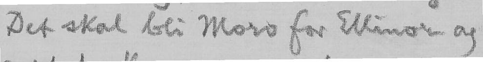Det skal bli Moro for Ellinor og
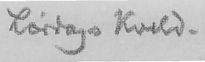Lørdags Kveld.
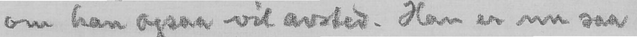om han ogsaa vil avsted. Han er nu saa
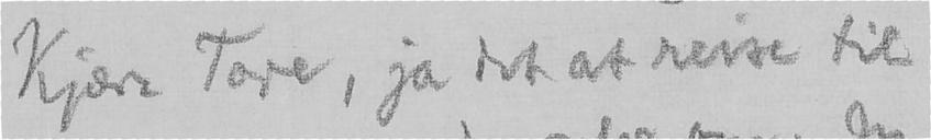Kjære Tore, ja det at reise til
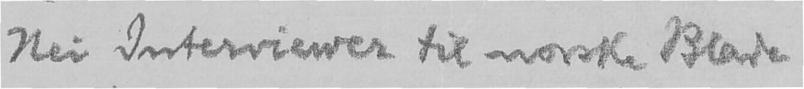Nei Interviewer til norske Blade
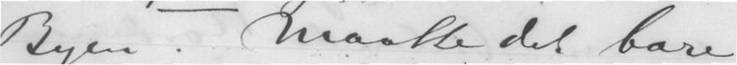Byen. Maatte det bare
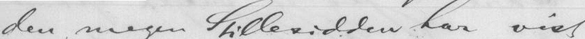den megen Stillesidden har vist
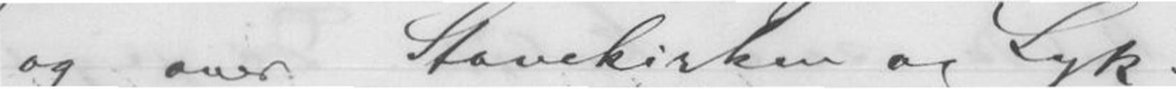og over Stavekirken og Lyk-
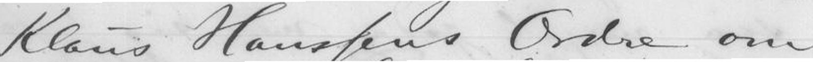Klaus Hanssens Ordre om
